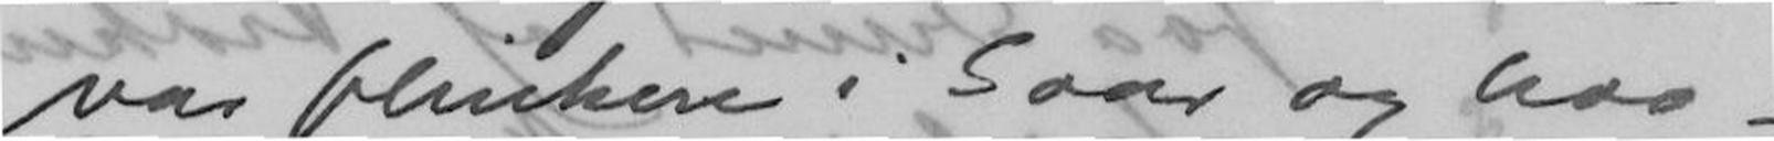var flinkere i Gaar og haa-
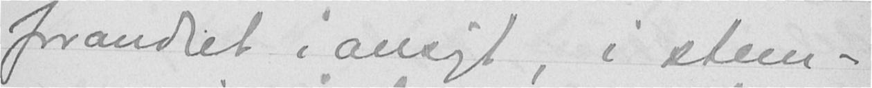forandret i ansigt, i stem-
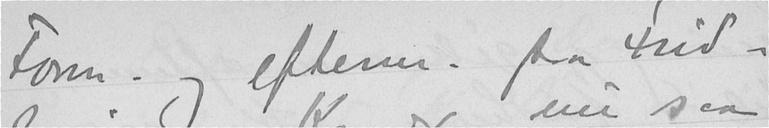Form. og efterm. paa Frid-
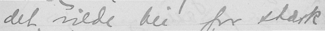det vilde bli for stærk
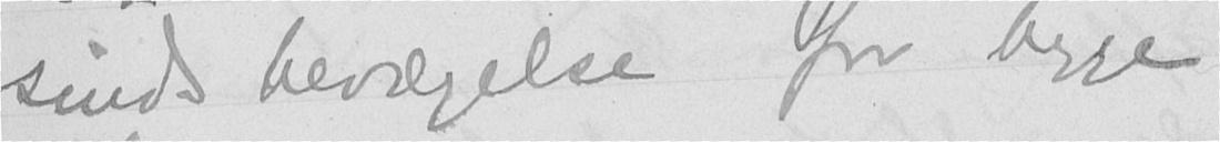sinds bevægelse for begge
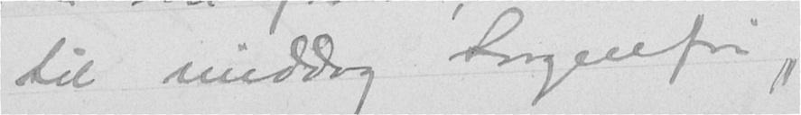til middag Sorgenfri,
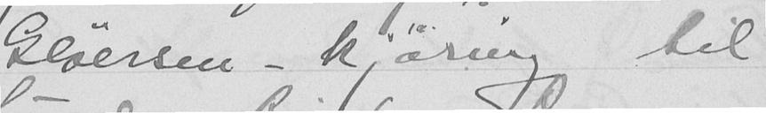Gløersen - kjøring til
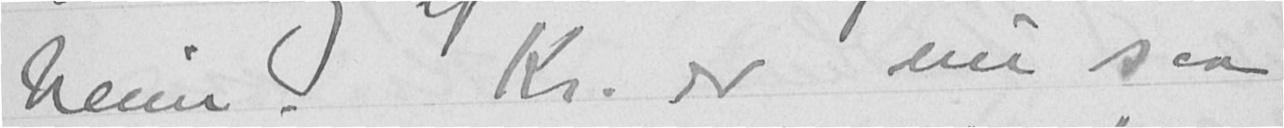heim. Kr. er nu saa
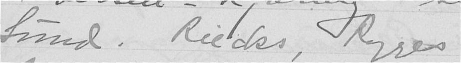Sund. Riecks, Rogges
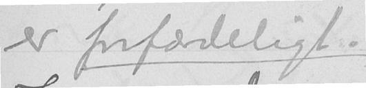er forfærdeligt.
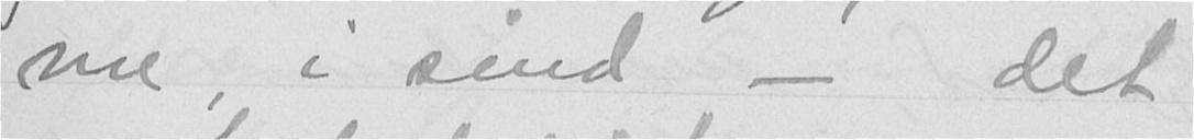me, i sind - det
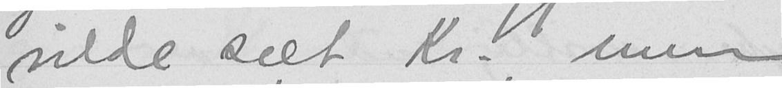vilde seet Kr. men
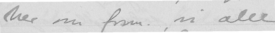her om form., vi alle
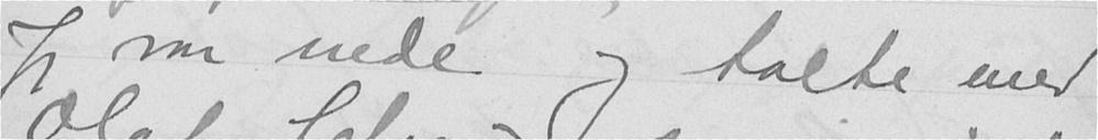Jeg var nede og talte med
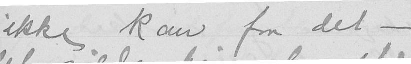ikke kan faa det -
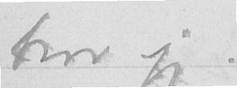tror jeg.
